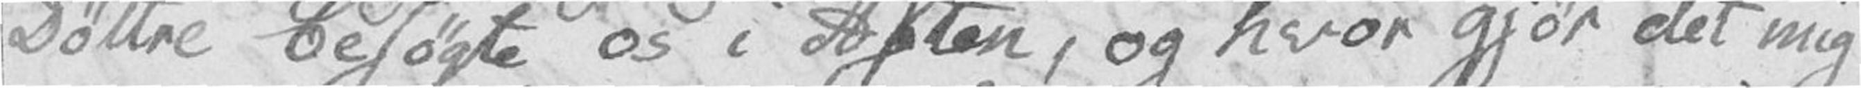Døttre besøgte os i Aften, og hvor gjør det mig
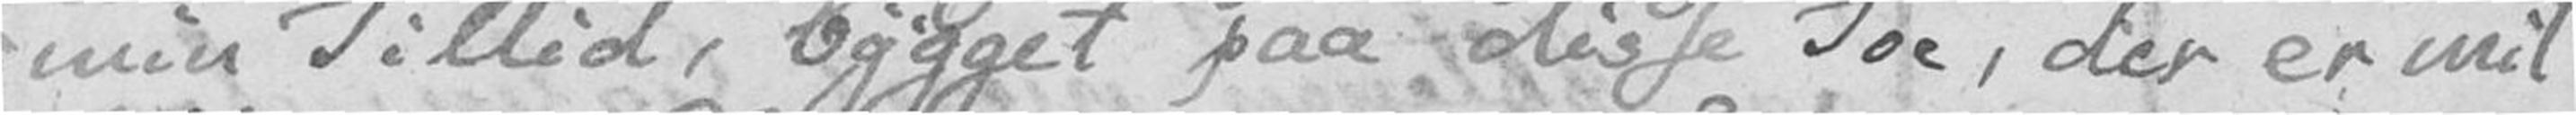min Tillid, bygget paa disse Toe, der er mit
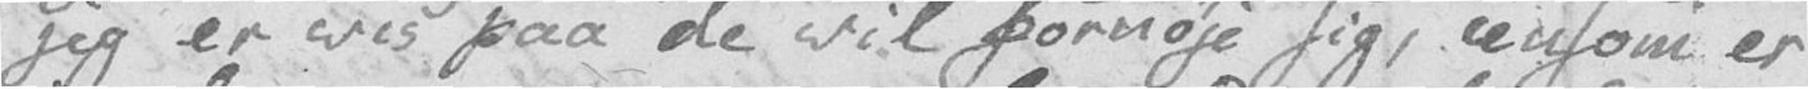jeg er vis paa de vil fornøje sig, eensom er
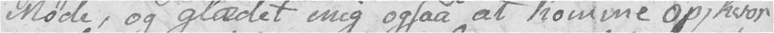Møde, og glædet mig ogsaa at komme op, hvor
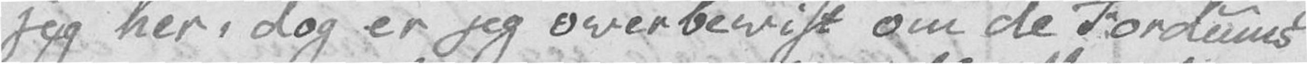jeg her, dog er jeg overbevist om de Fordums
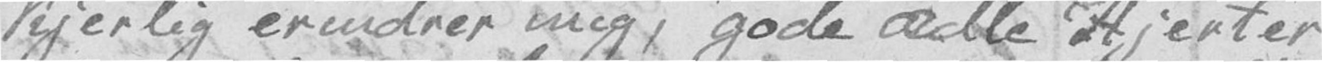kjerlig erindrer mig, gode ædle Hjerter
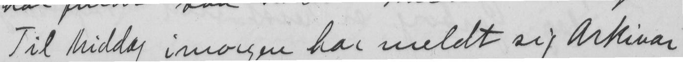Til Middag imorgen har meldt sig Arkivar
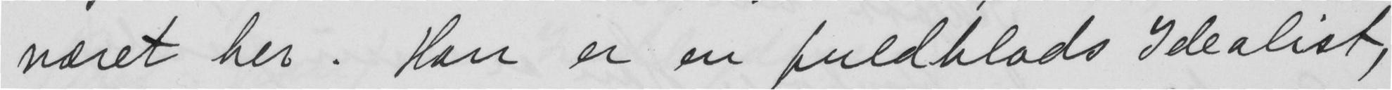været her. Han er en fuldblods Idealist,
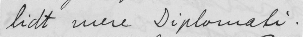lidt mere Diplomati.
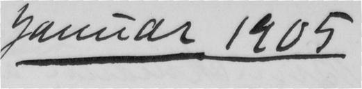Januar 1905
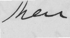Men
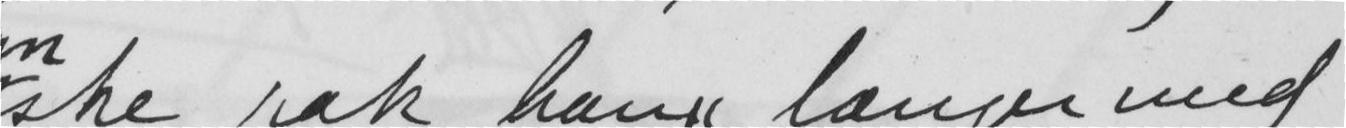ske ræk han længer med
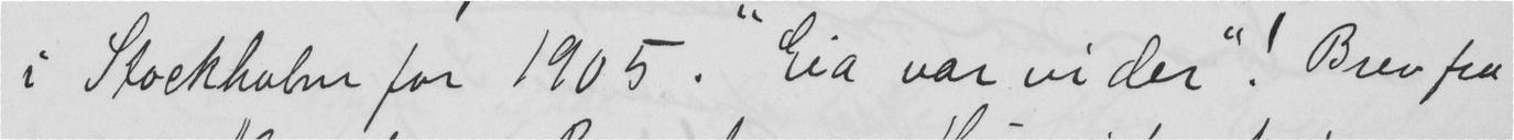i Stockholm for 1905. "Eia var vi der"! Brev fra
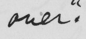over."
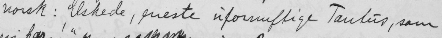norsk: "Elskede, eneste ufornuftige Tantus, som
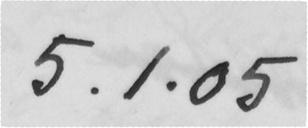5.1.05
